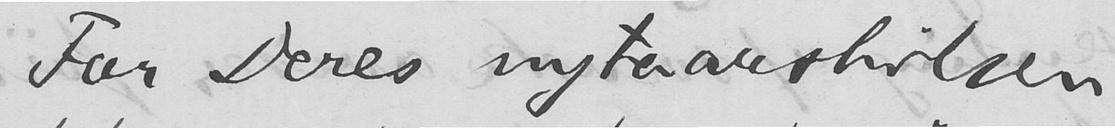For Deres nytaarshilsen
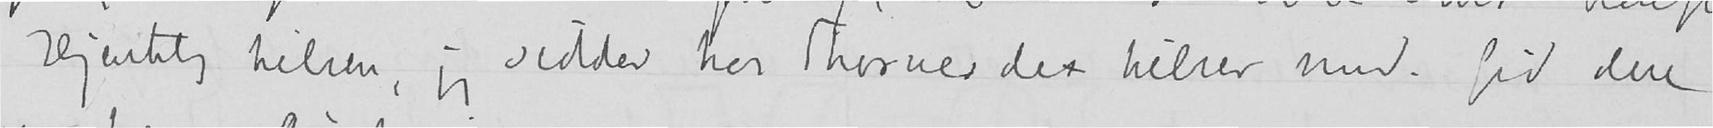Hjertelig hilsen, jeg sidder hos Thornes der hilser med. Gid dere
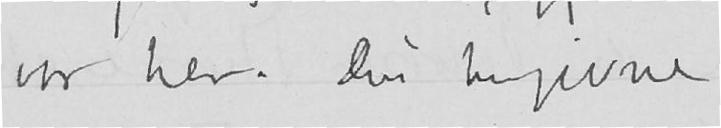var her. Din hengivne
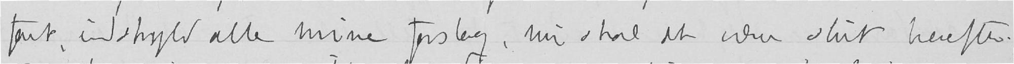fart, undskyld alle mine forslag, nu skal det være slut herefter.
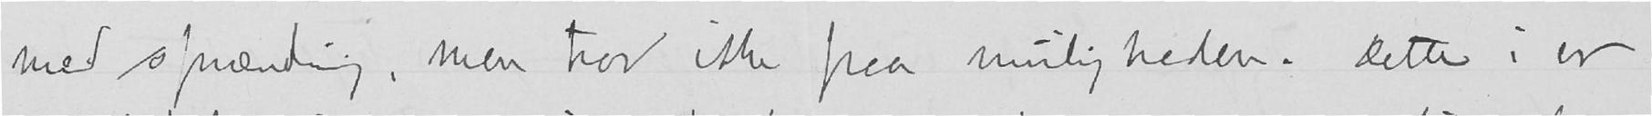med spænding, men tror ikke paa muligheden. Dette i en
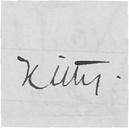Kitty.
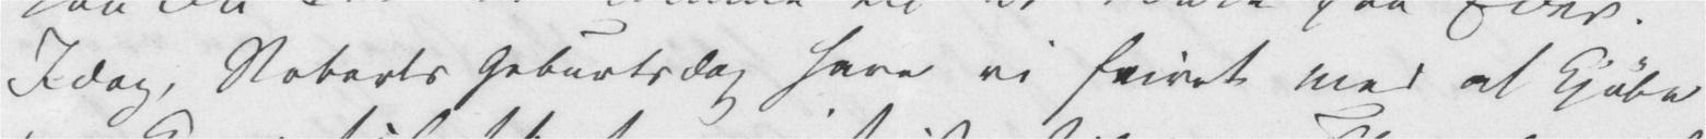Idag, Roberts Geburtsdag have vi feiret med at kjøbe
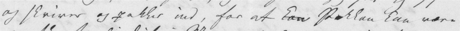og skriver og pakker ind, for at kan Pakken kan være
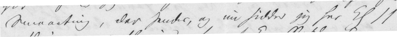Smaaeting, der sendes, og nu sidder jeg her Kl 11
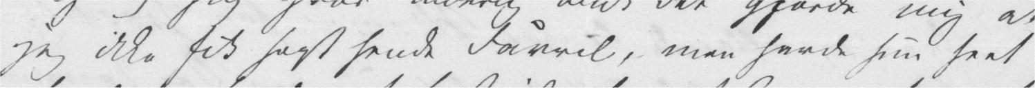jeg ikke fik sagt hende Farvel, men havde hun seet
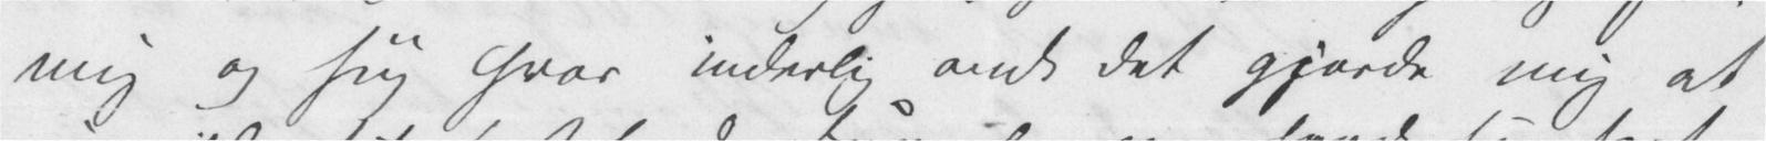mig og siig hvor inderlig ondt det gjorde mig at
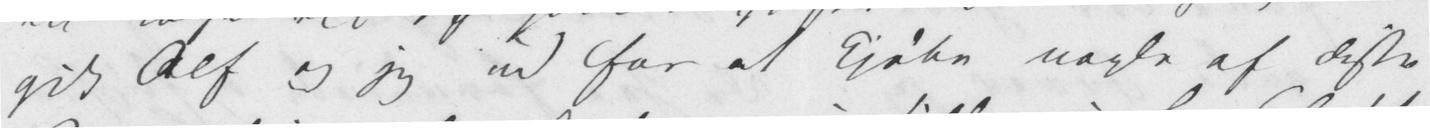gik Alf og jeg ud for at kjøbe nogle af disse
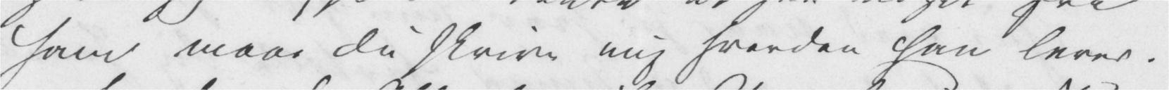ham maae Du skrive mig hvordan han lever.
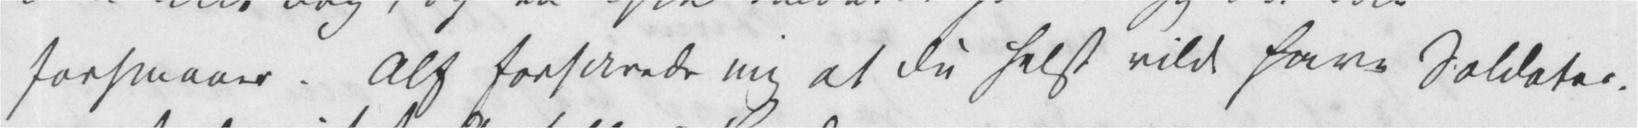forsmaaer. Alf forsikrede mig at Du helst vilde have Soldater.
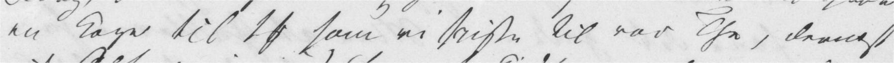en Kage til 1 [Ort] som vi spiste til vor The, dernæst
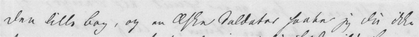Den lille Bog, og en Æske Soldater haaber jeg Du ikke
